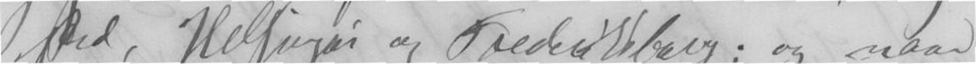sted, Helsingør og Fredriksborg; og naar
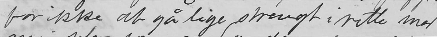for ikke at gå lige strengt i rette med
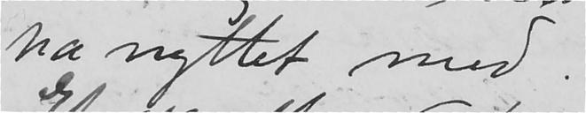ha nyttet med.
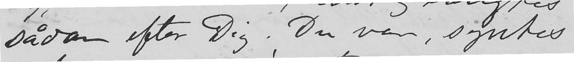sådan efter Dig. Du var, syntes
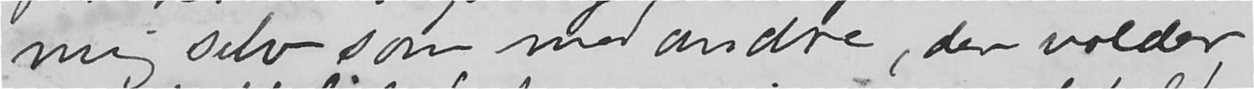mig selv som med andre, der volder
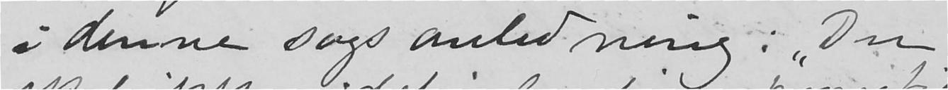i denne sags anledning: "Du
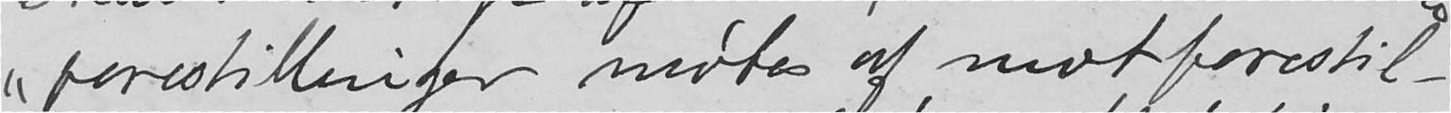"forestillinger møtes af motforestil-
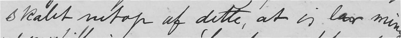skabt netop af dette, at og lar mine
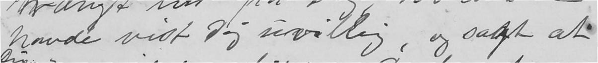havde vist Dig uvillig, og sagt at
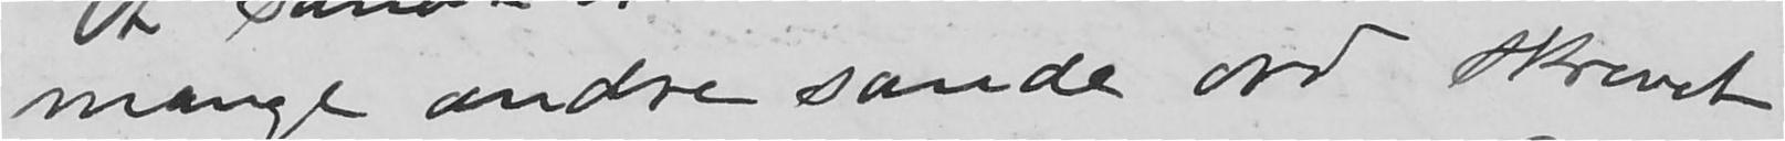mange andre sande ord skrevet
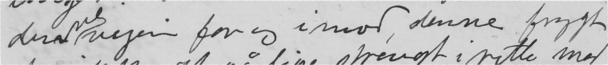denne vejen for og imod, denne frygt
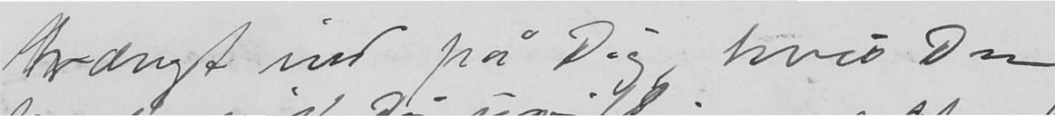trængt ind på Dig hvis Du
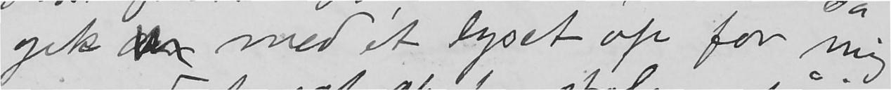gik der med ét lyset op for mig
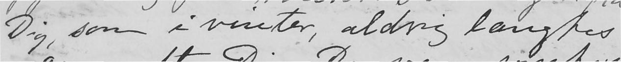Dig, som i vinter, aldrig længtes
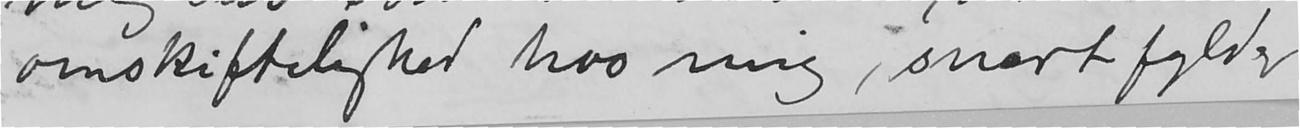omskiftelighed hos mig, snart fylder
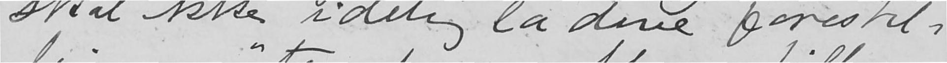skal ikke idelig la dine forestil-
